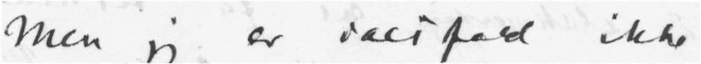Men jeg er ialtfald ikke
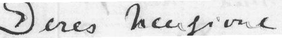Deres hengivne
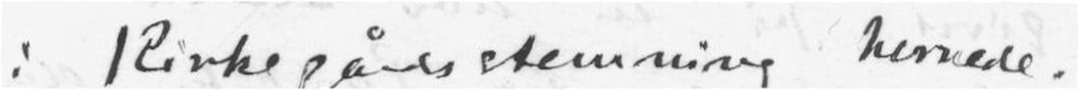i Kirkegårdstemning hernede.
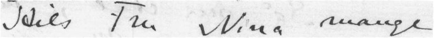Hils Fru Nina mange
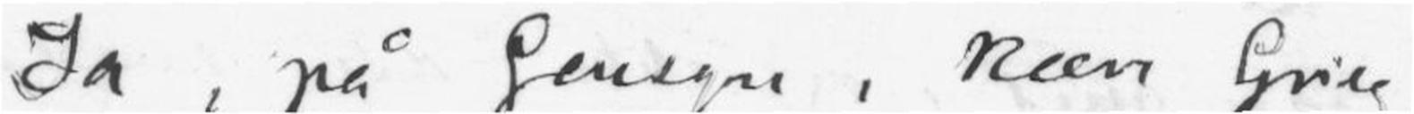Ja, på Gensyn, kære Grieg,
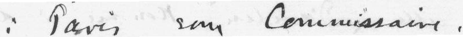i Paris som Commessaire,
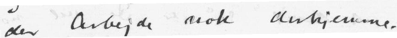der Arbejde nok derhjemme.
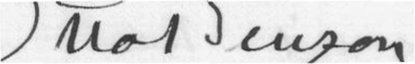Otto Benzon.
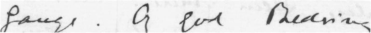Gange. Og god Bedring
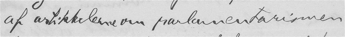af artikkelerne om parlamentarismen
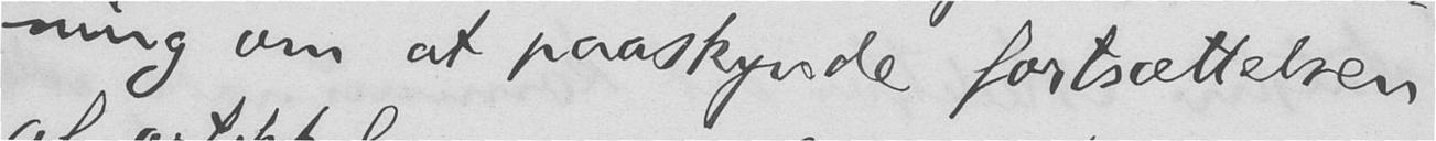ning om at paaskynde fortsættelsen
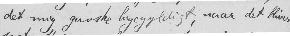det mig ganske ligegyldigt, naar det bliver
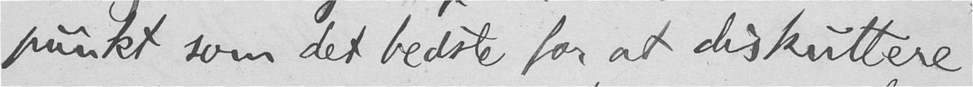punkt som det bedste for at diskuttere
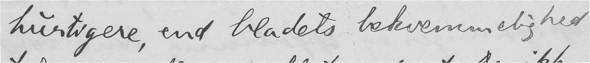hurtigere, end bladets bekvemmelighed
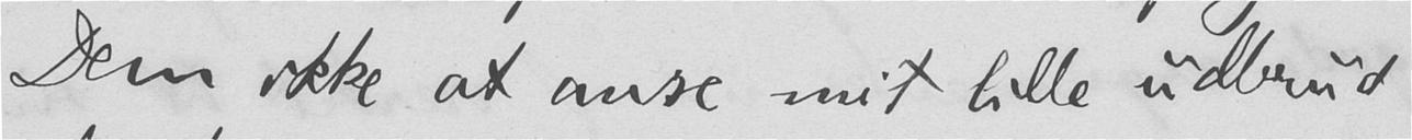Dem ikke at anse mit lille udbrud
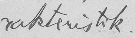rakteristik.
